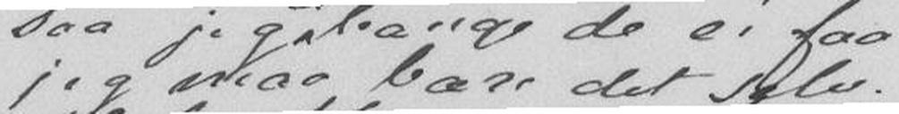jeg maa bære det selv.
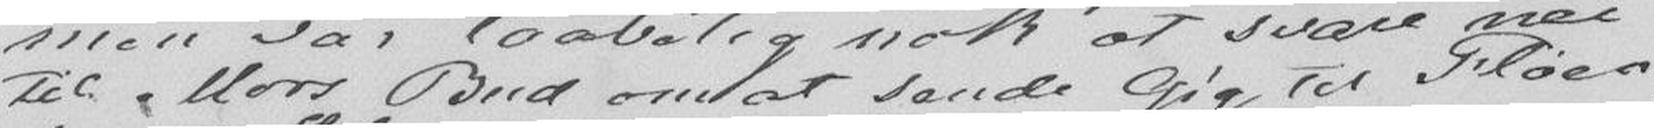til Mors Bud om at sende Gig til Fløen,
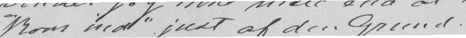"kom ind" just af den Grund.
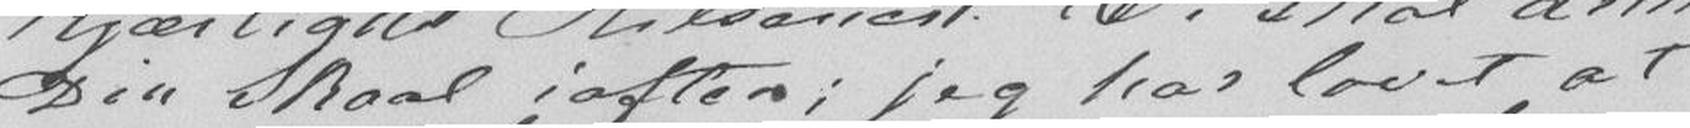Din Skaal iaften; jeg har lovet at
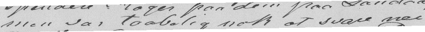man var taabelig nok at svare nei
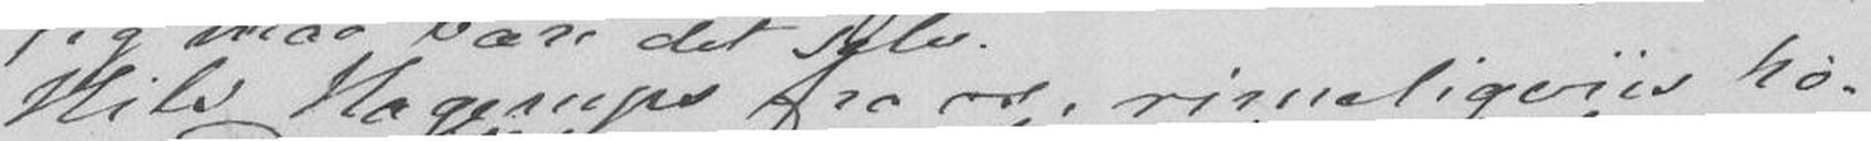Hils Hagerups fra os rimeligviis hø-
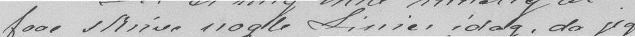faae skrive nogle Linier idag, da jeg
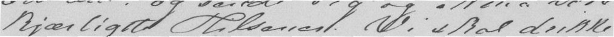kjæligste Hilsner. Vi skal drikke
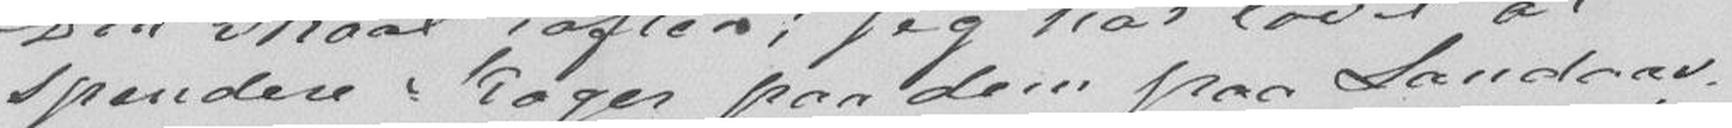spandere Kager paa dem paa Landaas,
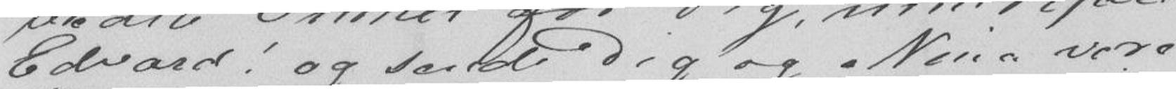Edvard! og sende Dig og Nina vore
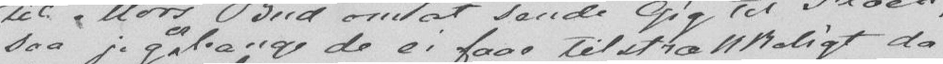saa jeg er bange de ei faae tilstrækkeligt da
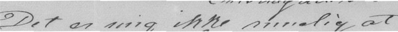Det er mig ikke muelig at
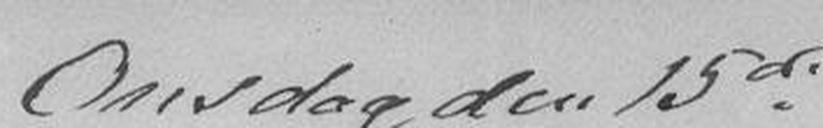Onsdag den 15de
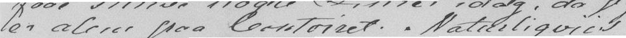er alene paa Contoiret. Naturligviis
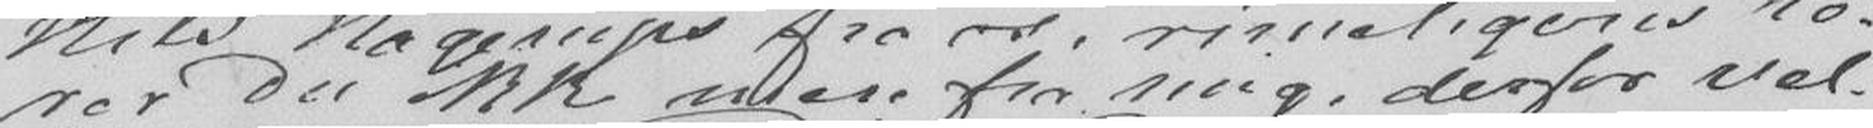rer Du ikke mere fra mig, derfor vel-
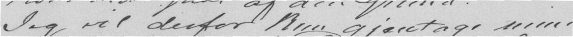Jeg vil derfor kun gjæntage mine
